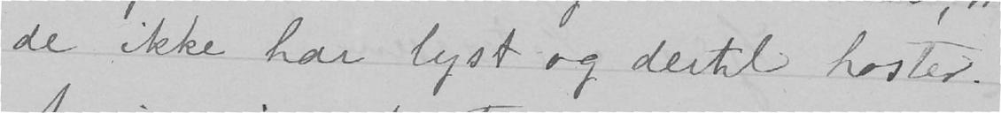de ikke har lyst og dertil hoster.
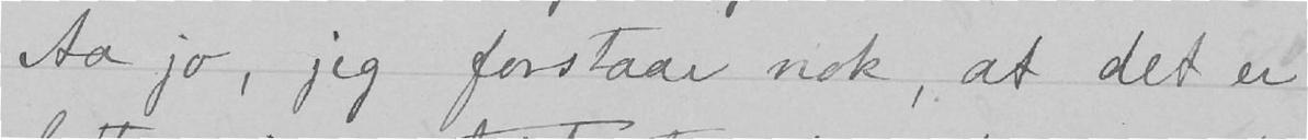Aa jo, jeg forstaar nok, at det er
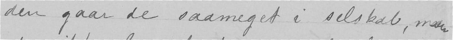den gaar de saameget i selskab, naar
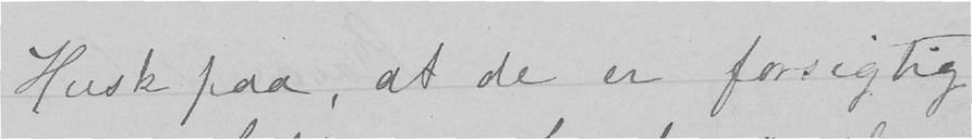Husk paa, at de er forsigtig
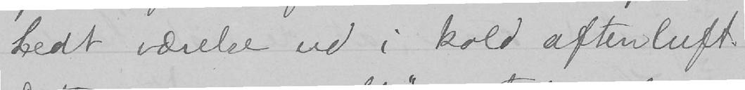hedt værelse ud i kold aftenluft.
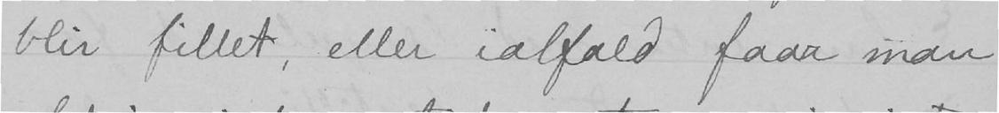blir fillet, eller ialfald faar man
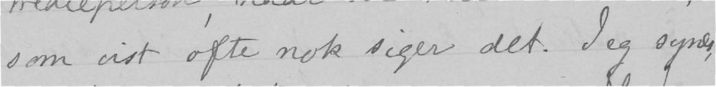som vist ofte nok siger det. Jeg synes,
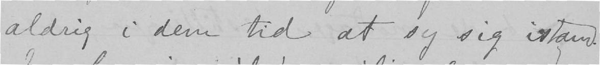aldrig i dem tid at sy sig istand.
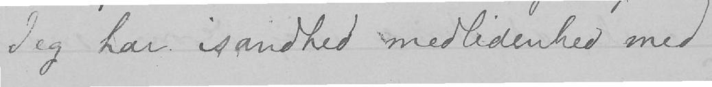Jeg har isandhed medlidenhed med
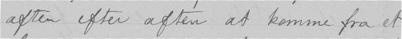aften efter aften at komme fra et
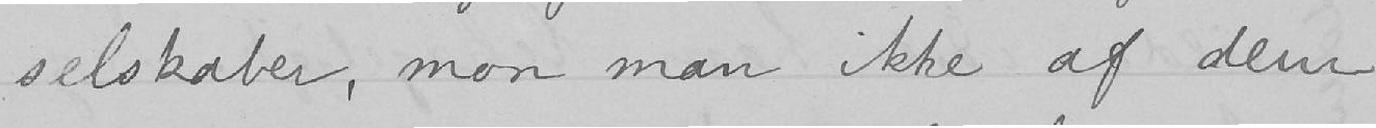selskaber, mon man ikke af dem
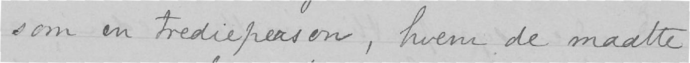som en tredieperson, hvem de maatte
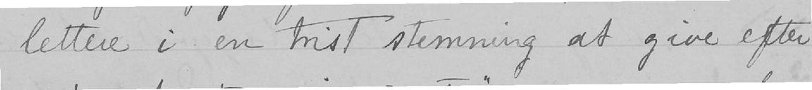lettere i en trist stemning at give efter
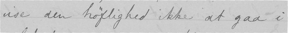vise den høflighed ikke at gaa i
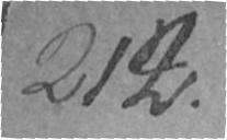212.
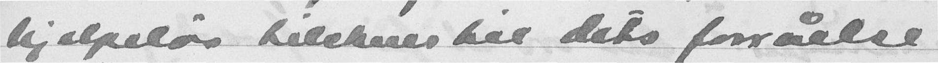hjelpeløs tilskuer til dets forråelse
